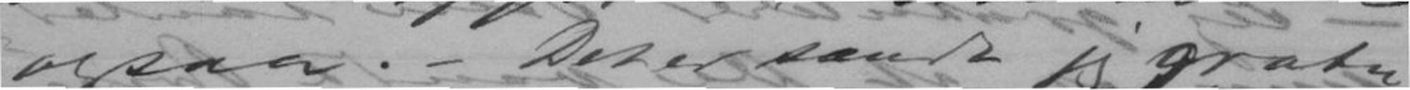ogsaa. - Det er sandt jeg gratu-
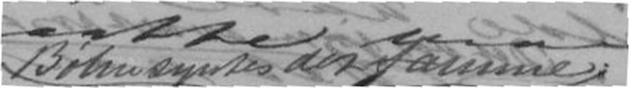Bøhn syntes det samme,
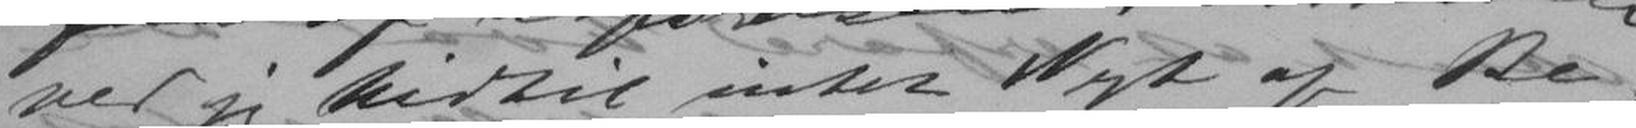ved jeg hidtil intet Nyt af Be-
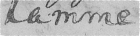Lamme
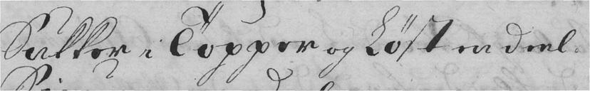Sukker i Topper og Løst en deel.
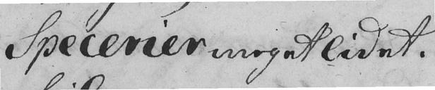Specerier meget lidet.
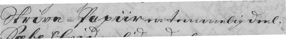Skrive-Papiir en temmelig deel.
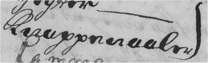Knappenaaler
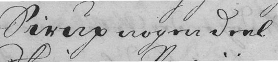Sirup nogen deel
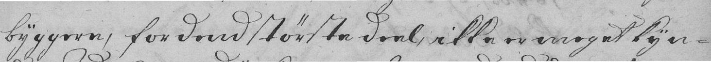byggere, for dend største deel, ikke er meget kyn-
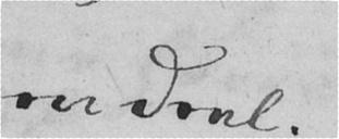en deel.
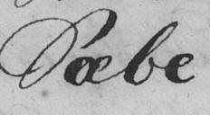Sæbe
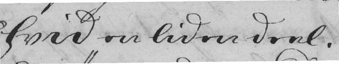hvid en liden deel.
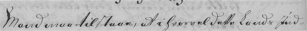Mand maa tilstaae, at i hvorvel dette Lands Ind-
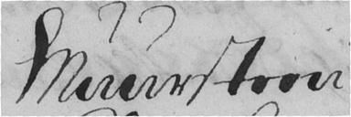Muursteen
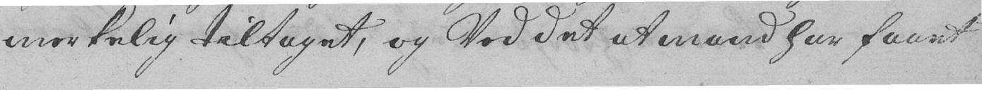merkelig tiltaget, og Ved det at mand har faaet
